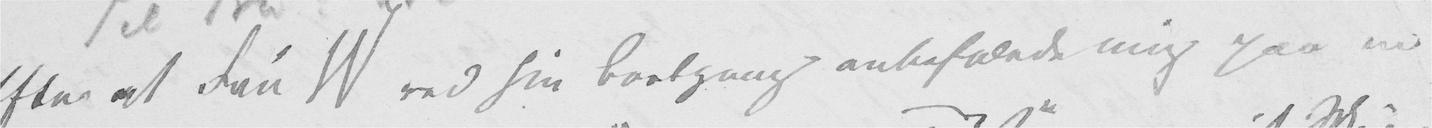Efter at Fru W ved sin Bortgang anbefalede mig paa en
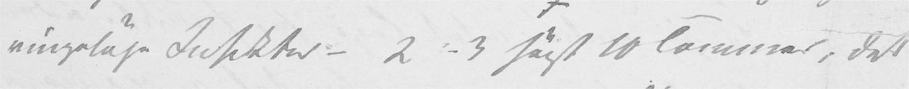vingeløse Insekter – 2–3 høist 10 Tommer, det
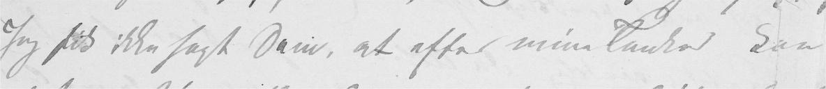Jeg fik ikke sagt Dem, at efter mine Tanker kan
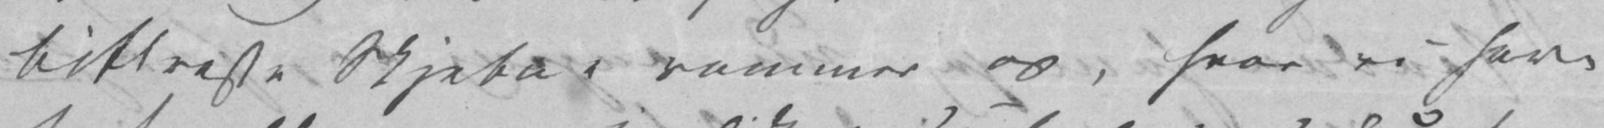bittreste Skjebne rammer os, hvor vi have
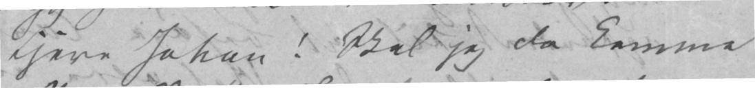Kjere Johan! Skal jeg da komme
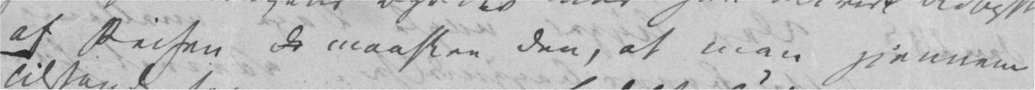af Reisen d maaskee den, at man gjennem
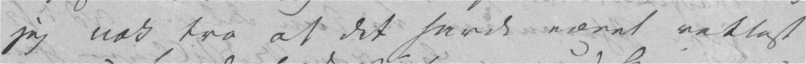jeg nok tro at det havde været rettest
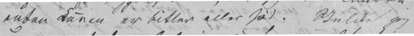enten Kuren er bitter eller sød. Skulde jeg
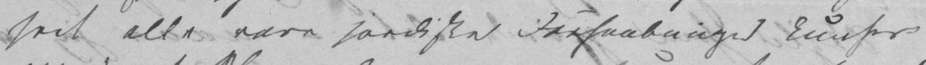seet alle vore jordiske Forhaabninger knuses
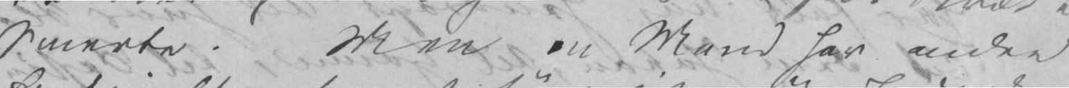Smerte. Men en Mand har andre
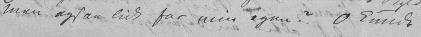men ogsaa lidt for min egen? O kunde
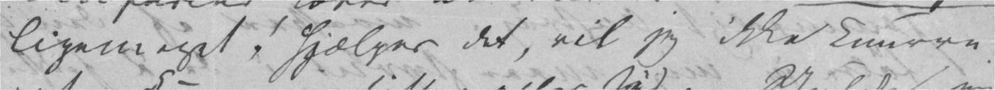ligemeget! Hjælper det, vil jeg ikke knurre
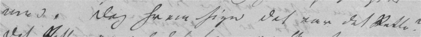med. Dog hvem siger det var det Rette?
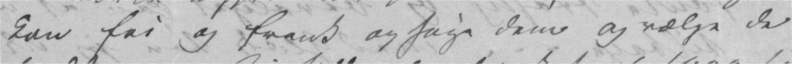kan fri og frank opsøge dem og vælge de
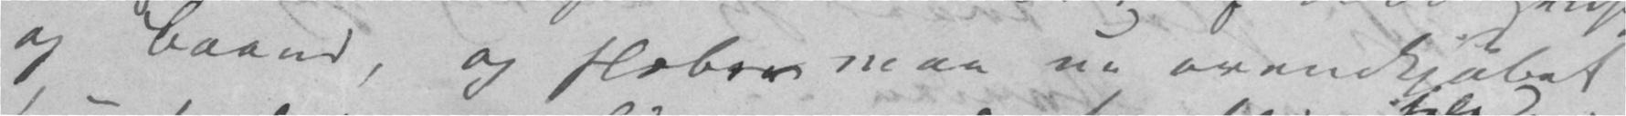og Baand, og slæber man nu ovenikjøbet
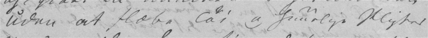uden at slæbe Tøi og huuslige Pligter
